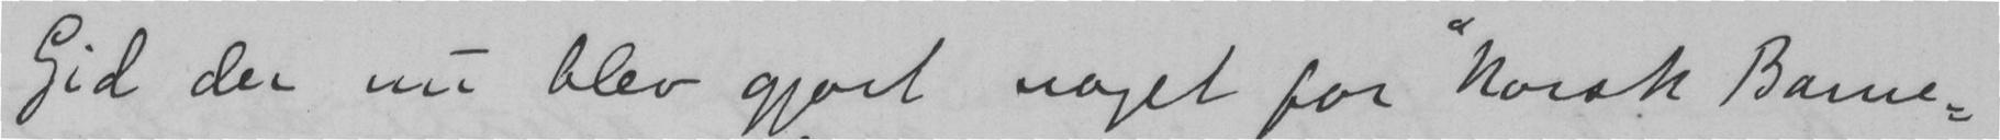Gid der nu blev gjort noget for "Norsk Barne-
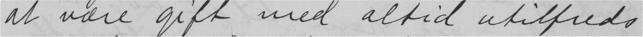at være gift med altid utilfreds
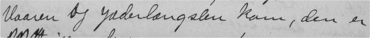Vaaren og Jæderlængslen kom, den er
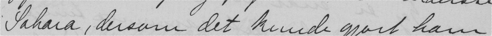Sahara, dersom det kunde gjort ham
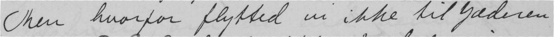Men hvorfor flytted vi ikke til Jæderen
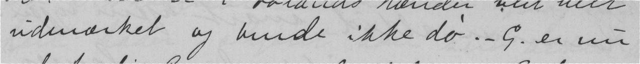udmærket og burde ikke dø. - G. er nu
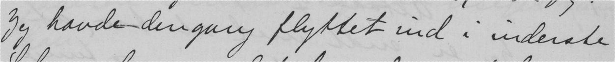Jeg havde dengang flyttet ind i inderste
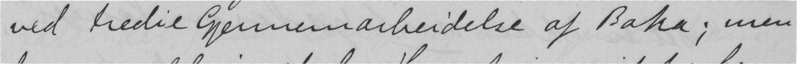ved tredie Gjennemarbeidelse af Boka; men
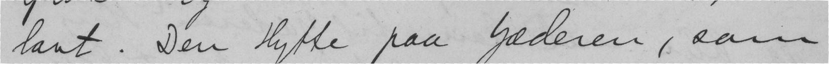lavt. Den Hytte paa Jæderen, som
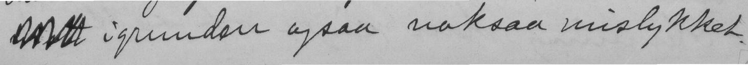igrunden ogsaa noksaa mislykket.
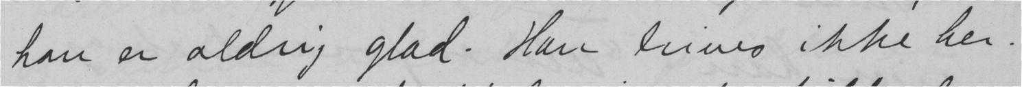han er aldrig glad. Han trives ikke her.
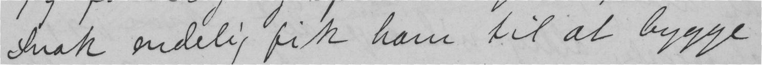Snak endelig fik ham til at bygge
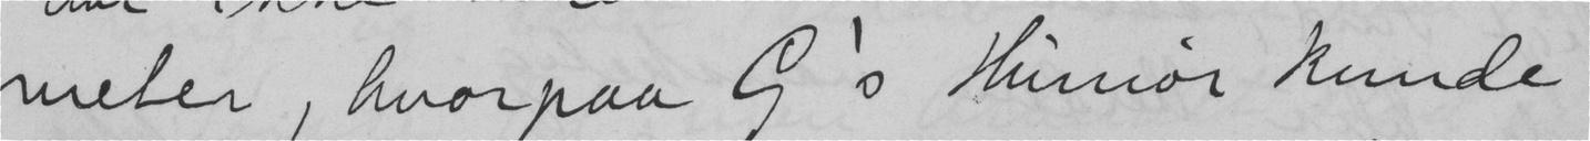meter, hvorpaa G's Humør kunde
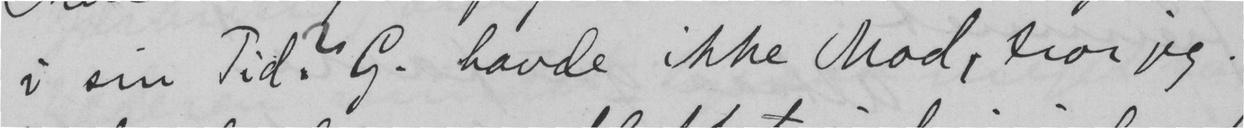i sin Tid? G. havde ikke Mod, tror jeg.
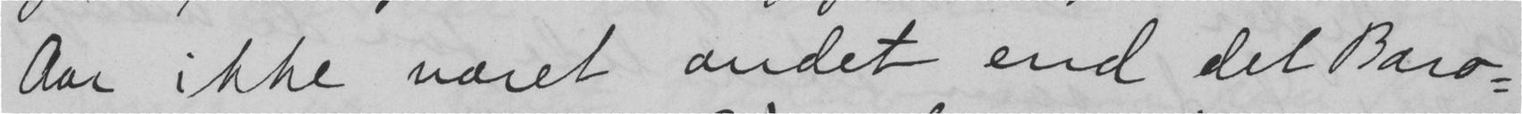Aar ikke været andet end det Baro-
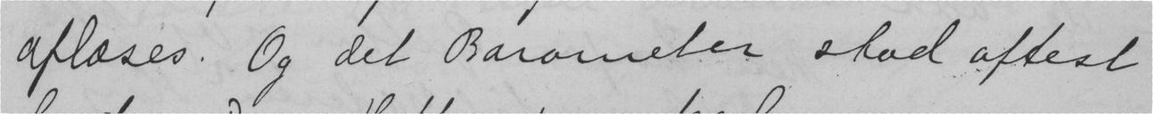aflæses. Og det Barometer stod oftest
